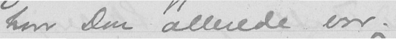hvor Don allerede var.
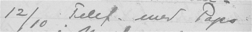12/10 Telef. med Paps
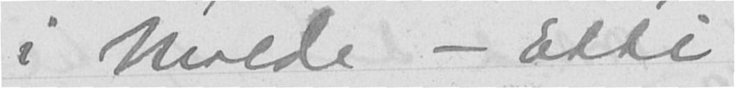i Molde - Etti
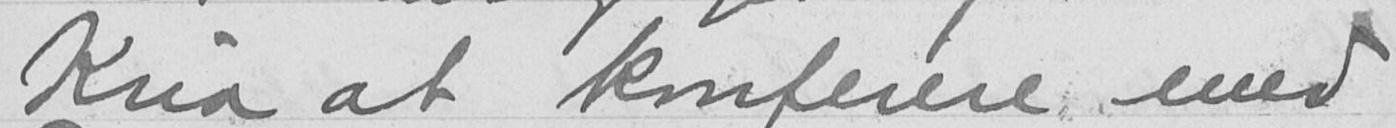Kria at konferere med
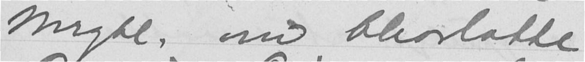Mrgbl, om Charlotte
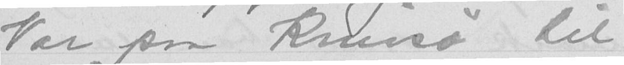Var paa Knivsø til
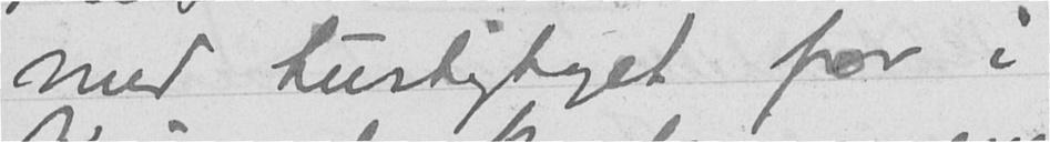med hurtigtoget for i
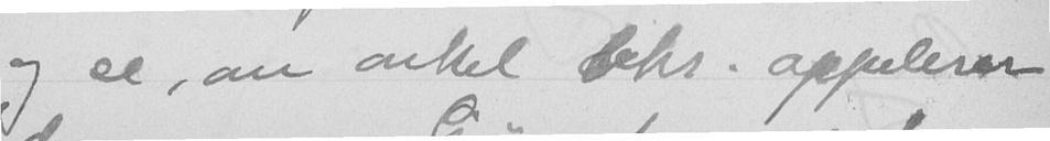og se, om onkel Chr. appelerer
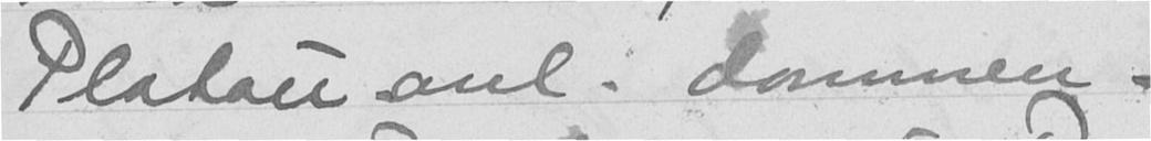Platou anl. dommen.
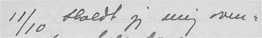11/10 Holdt jeg mig oven-
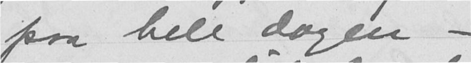paa hele dagen -
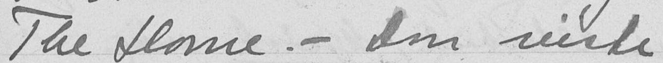The Home. - Don reiste
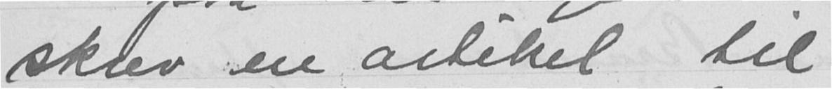skrev en artikel til
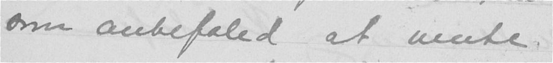som anbefaled at vente
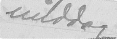middag.
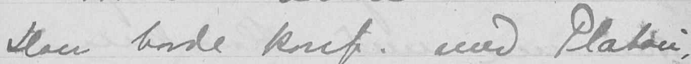Han havde konf. med Platou,
